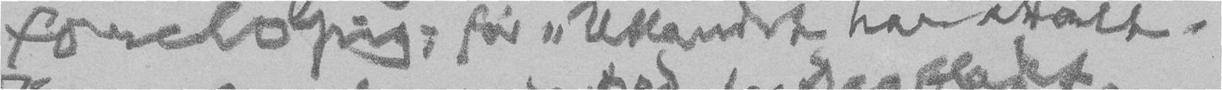foreløpig; før "Utlandet har uttalt.
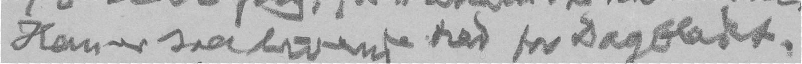Han er saa livende red for Dagbladet,
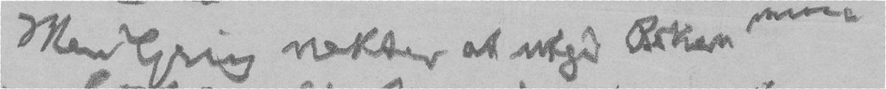Men Grieg nekter at utgi Boken min
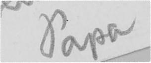Papa
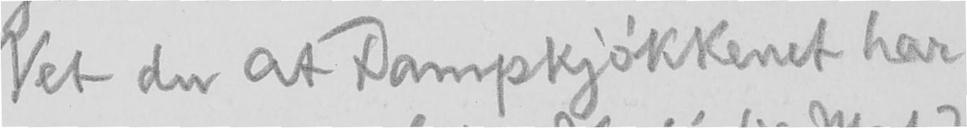Vet du at Dampkjøkkenet har
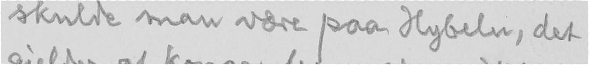skulde man være paa Hybeln, det
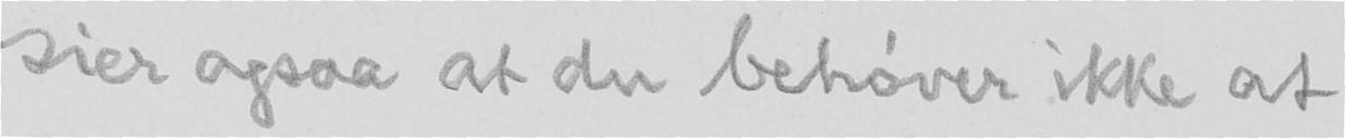sier ogsaa at du behøver ikke at
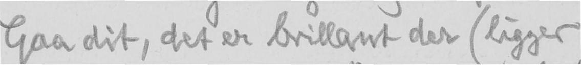Gaa dit, det er brillant der (ligger
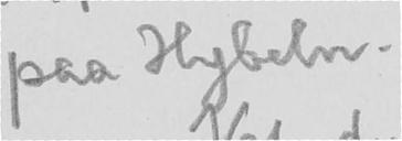paa Hybeln.
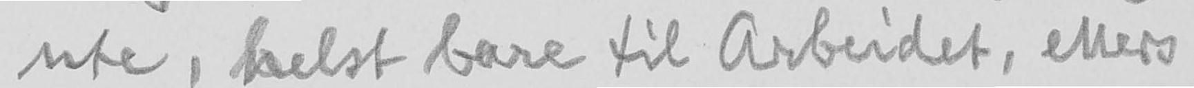ute, helst bare til Arbeidet, ellers
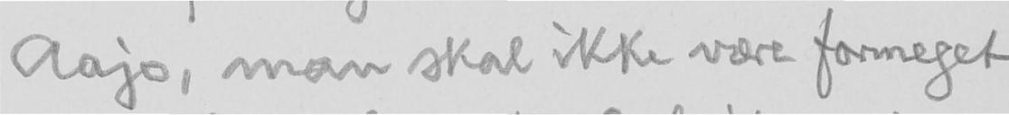Aajo, man skal ikke være formeget
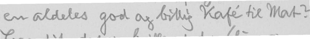en aldeles god og billig Kafé til Mat?
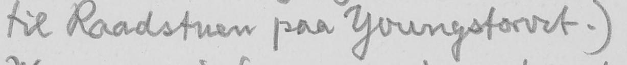til Raadstuen paa Youngstorvet.)
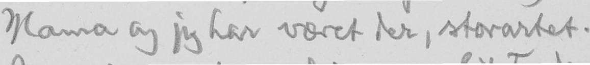Mama og jeg har været der, storartet.
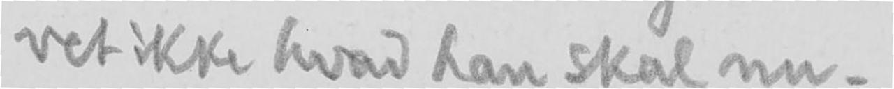vet ikke hvad han skal nu.
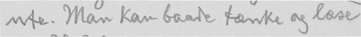ute. Man kan baade tænke og læse
